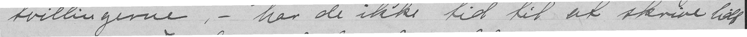tvillingerne ,- har de ikke tid til at skrive lidt
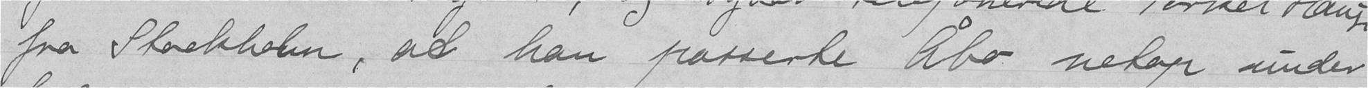fra Stockholm, at han passerte Åbo netop under
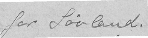for Løvland.
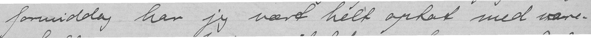formiddag har jeg vært helt optat med vare-
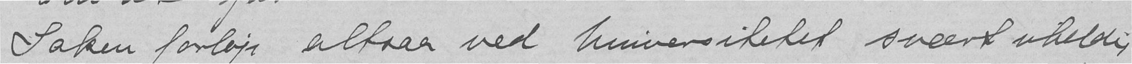Saken forløp altsaa ved Universitetet svært uheldig
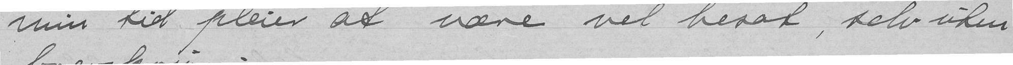min til pleier at være vel besat, selv uden
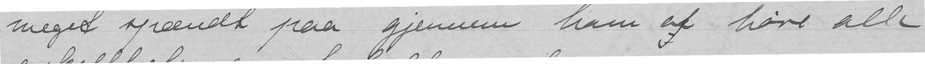meget spændt paa gjennem ham at høre alle
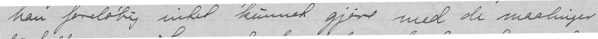han foreløbig intet kunnet gjøre med de maalinger
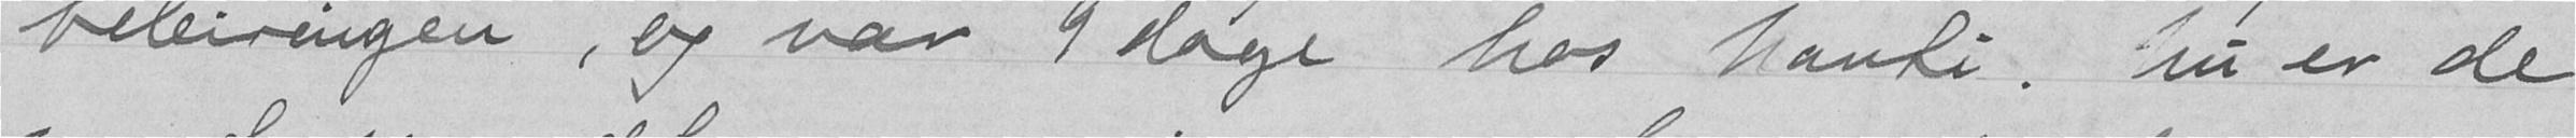beleringen, og var 9 dage hos Nanti. Nu er de
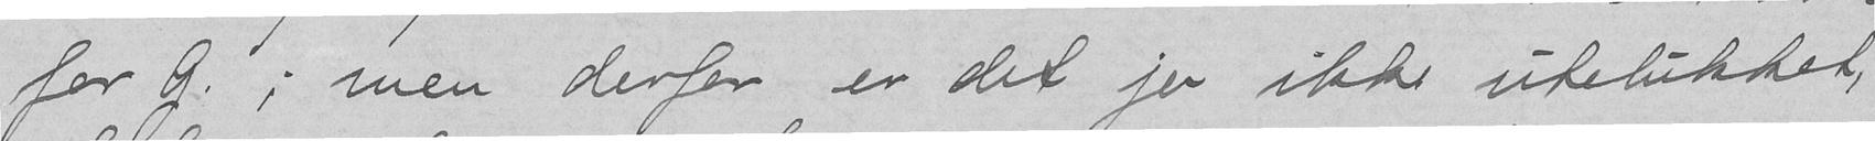for G.; men derfor er det jo ikke utelukket,
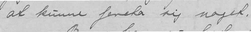at kunne foreta sig noget.
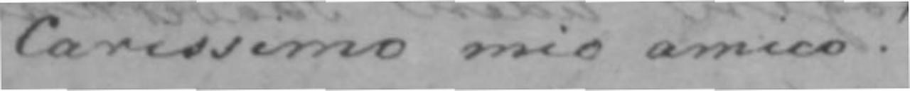Carissimo mio amico!
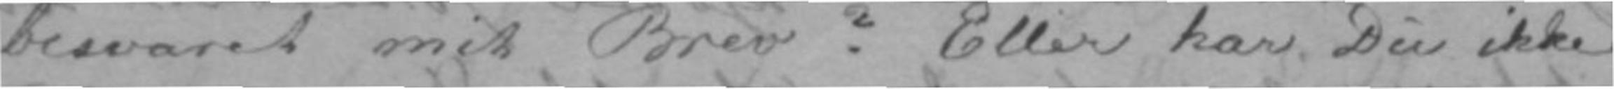besvaret mit Brev? Eller har Du ikke
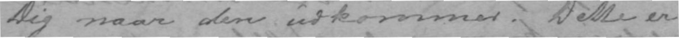Dig naar den udkommer. Dette er
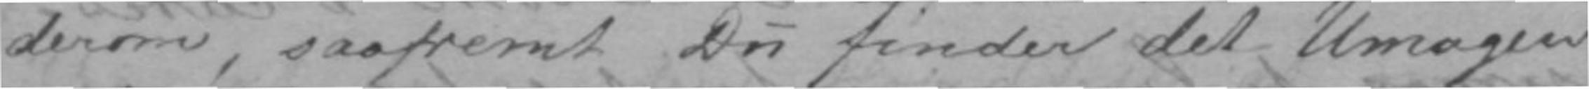derom, saafremt Du finder det Umagen
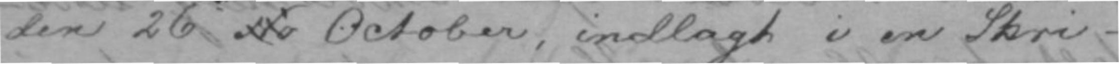den 26de No October, indlagt i en Skri-
